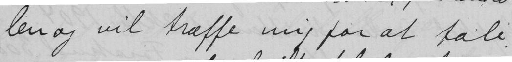len og vil træffe mig for at tale
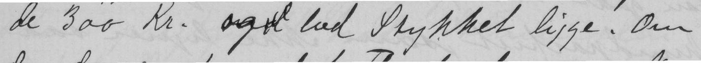de 300 Kr. og lod Stykket ligge. Om
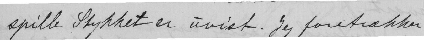spille Stykket er uvist. Jeg foretrækker
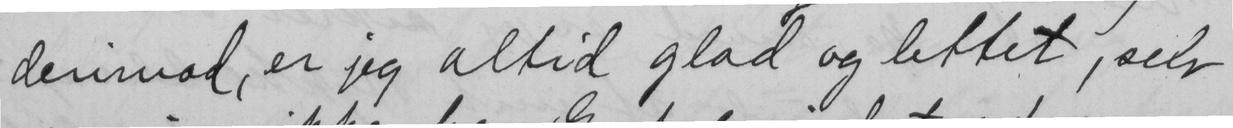derimod, er jeg altid glad og lettet, selv
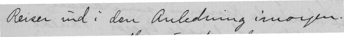Reiser ind i den Anledning imorgen.
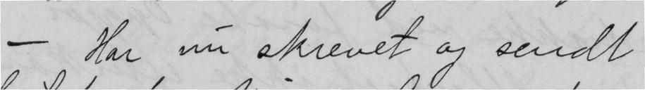- Har nu skrevet og sendt
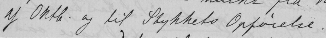af Oktb. og til Stykkets Opførelse.
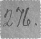276.
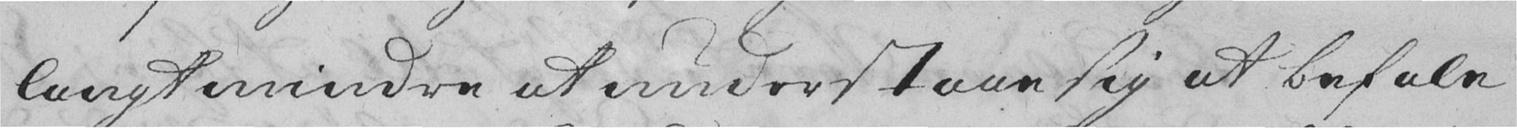langt mindre at understaae sig at befale
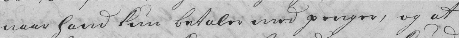naar hand kun betaler med penger, og at
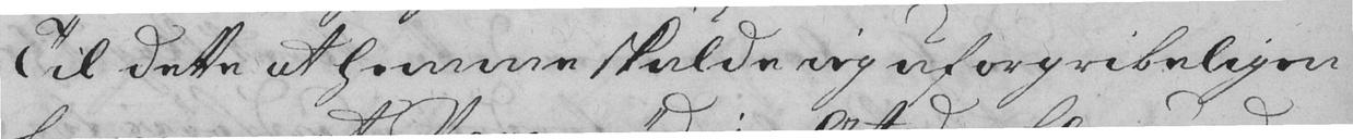Til dette at hemme skulde jeg uforgribeligen
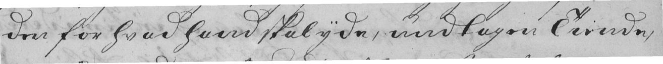den for hvad hand skal yde, undtagen Tiende,
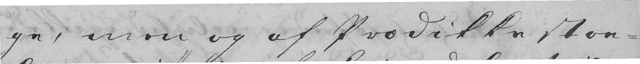ge, men og af Prædikkestoe-
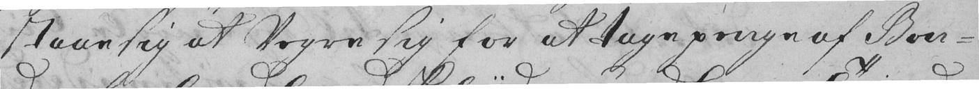staae sig at Vegre sig for at tage penge af Bon-
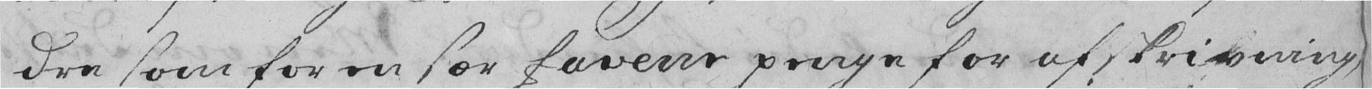dre som for en sær forene penge for afskrivning,
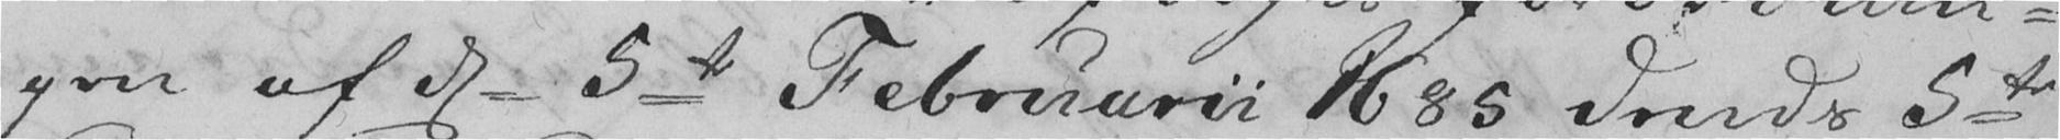gen af d 5te Februarii 1685 dends 5te
